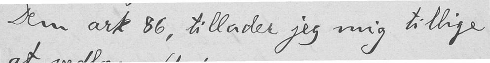Dem ark 86, tillader jeg mig tillige
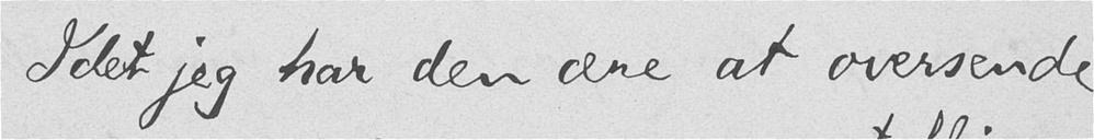Idet jeg har den ære at oversende
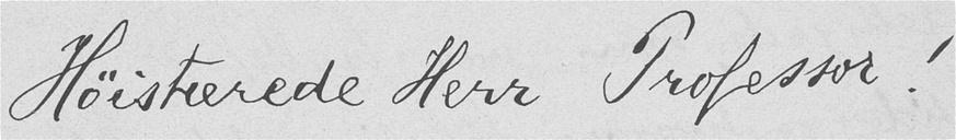Høistærede Herr Professor!
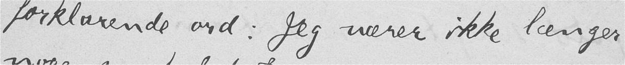forklarende ord: Jeg nærer ikke længer
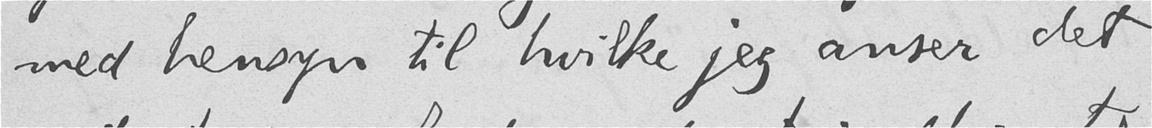med hensyn til hvilke jeg anser det
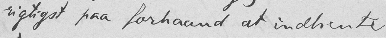rigtigst paa forhaand at indhente
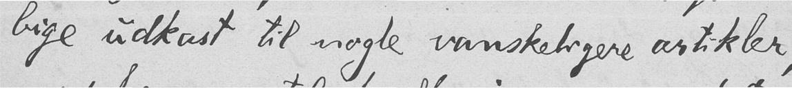bige udkast til nogle vanskeligere artikler,
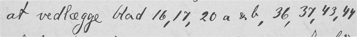at vedlægge blad 16, 17, 20 a & b, 36, 37, 43, 44
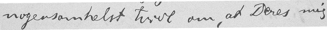nogensomhelst tvivl om, at Deres mig
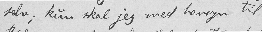selv; kun skal jeg med hensyn til
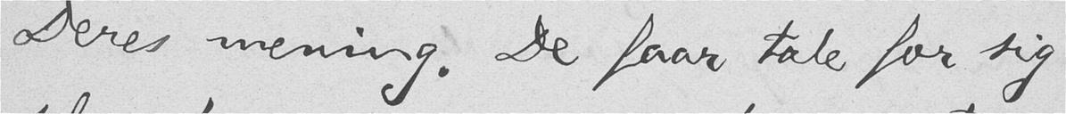Deres mening. De faar tale for sig
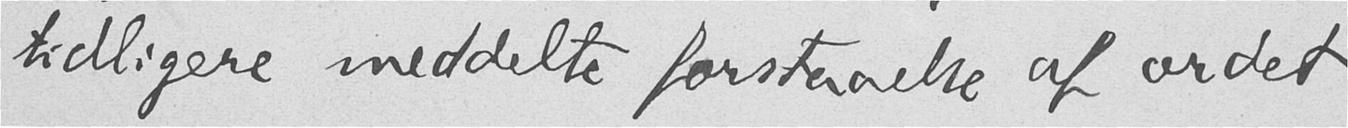tidligere meddelte forstaaelse af ordet
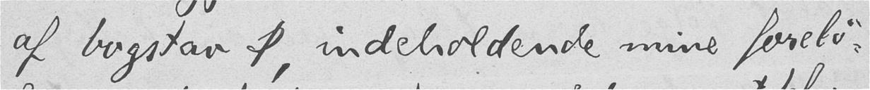af bogstav Þ, indeholdende mine forelø-
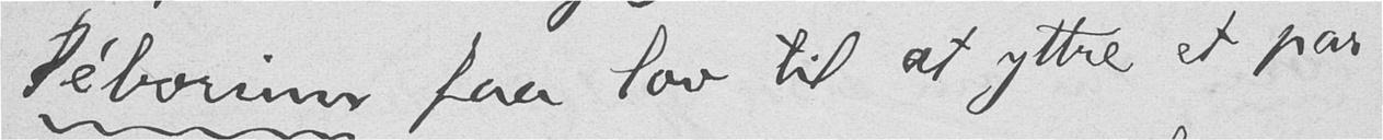Þéborinn faa lov til at yttre et par
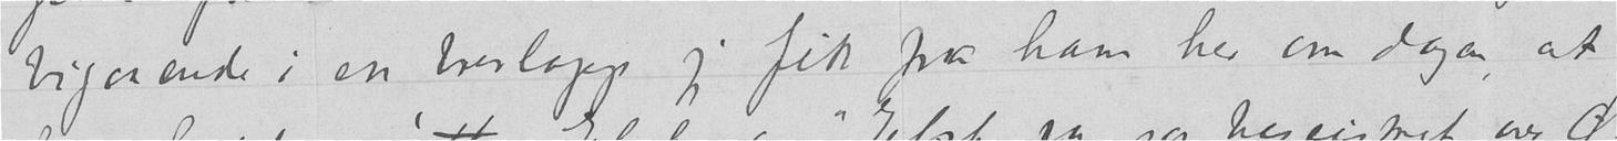bigaaende i en brevlapp jeg fik fra ham her om dagen, at
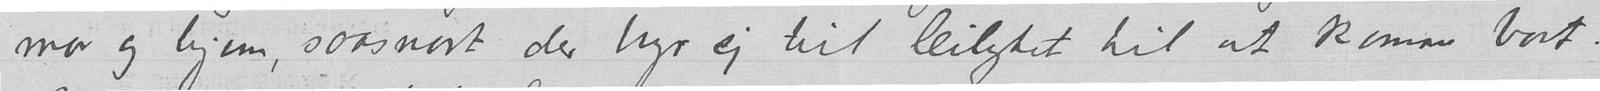mor og hjem, saasnart der byr sig til leilighet til at komme bort.
